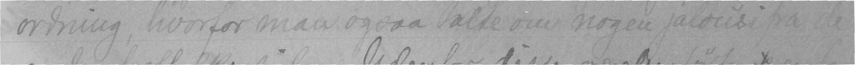ordning, hvorfor man ogsaa talde om nogen jalousi fra de
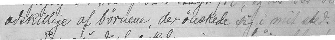adskillige af børnene, der ønskede sig i mit sted.
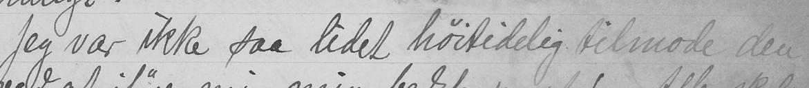Jeg var ikke saa lidet høitidelig tilmode den
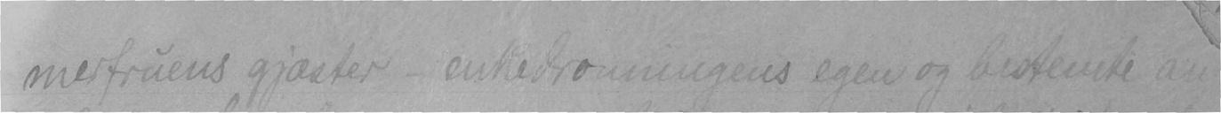merfruens gjæster - enkedronningens egen og bestemde an-
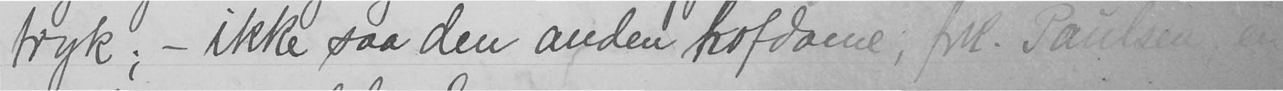tryk; - ikke saa den anden hofdame; frk. Paulsen, en
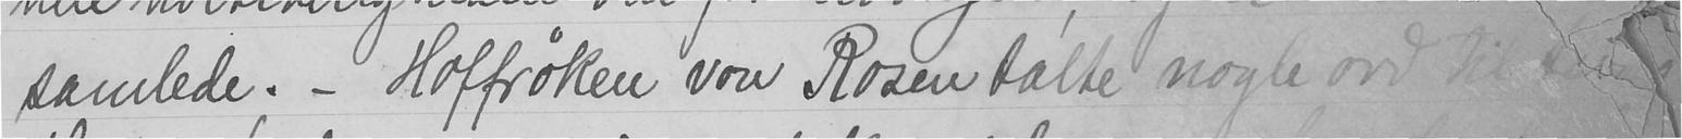samlede. - Hoffrøken von Rosen talte nogle ord til
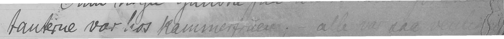tanterne var hos kammerfruen; alle var saa venlige
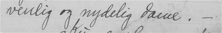venlig og nydelig dame. -
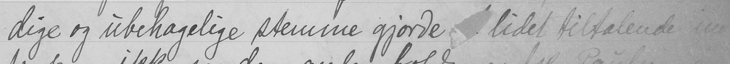dige og ubehagelige stemme gjorde et  lidet tiltalende ind-
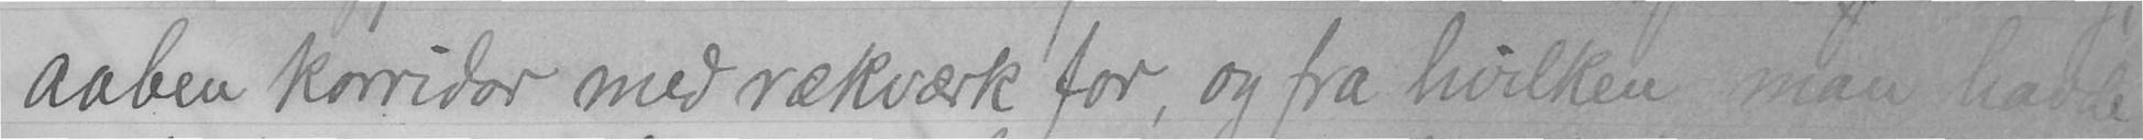aaben korridor med rækværk for, og fra hvilken man hadde
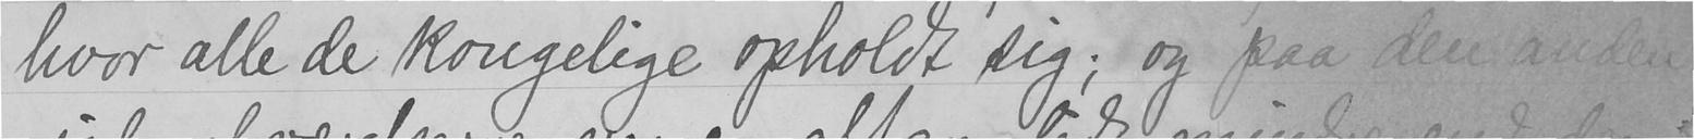hvor alle de kongelige opholdt sig; og paa den anden
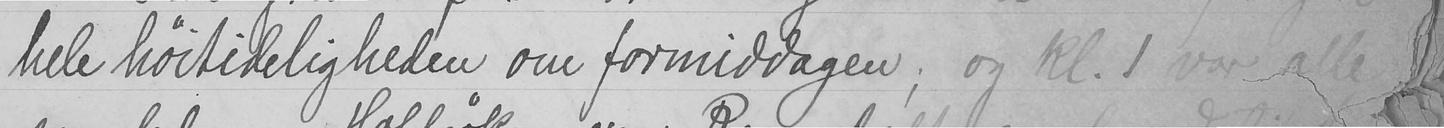hele høitideligheden om formiddagen; og kl. 1 var alle
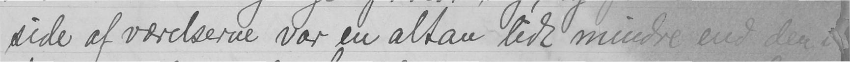side af værelserne var en altan lidt mindre end den i
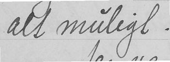alt muligt.
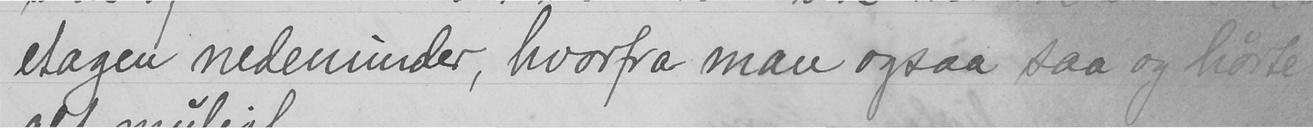etagen nedenunder, hvorfra man ogsaa saa og hørte
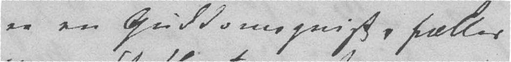er en Guddomsgnist, fælles
